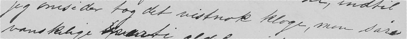jeg omsider tog det vistnok kloge, men såre
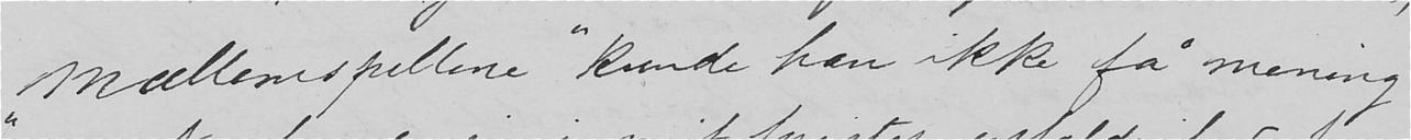"mællemspillene" kunde han ikke få mening
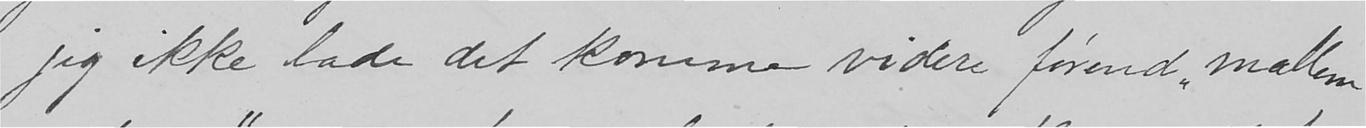jeg ikke lade det komme videre førend, "mællem-
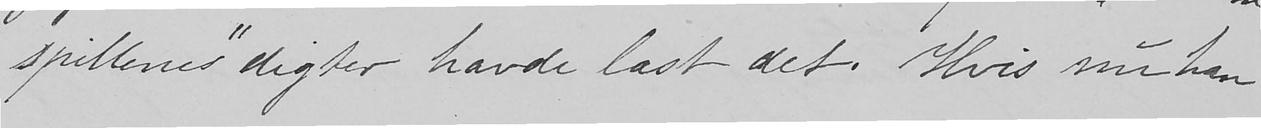spillenes" digter havde læst det. Hvis nu han
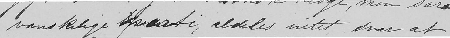vanskelige parti, aldeles intet svar at
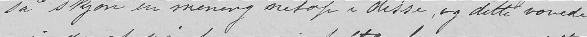så skjøn en mening netop i disse, og dette vovede
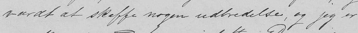værdt at skaffe nogen udbredelse, og jeg er
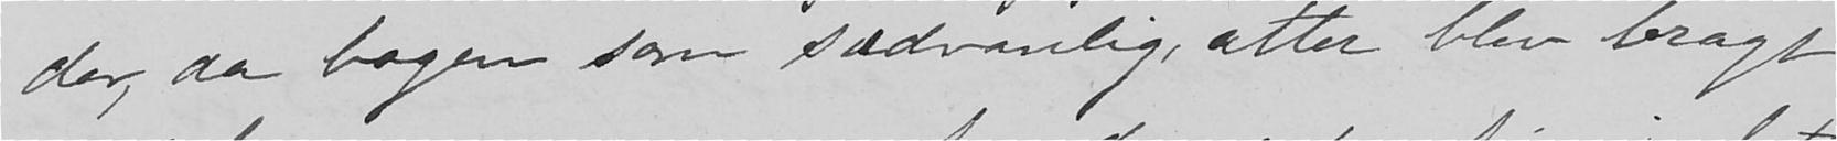der, da bogen som sædvanlig, atter blev bragt
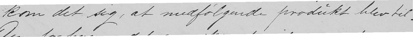kom det sig, at medfølgende produkt blev til.
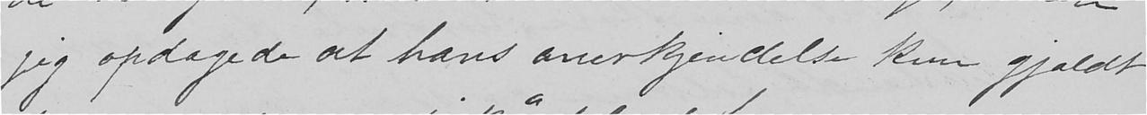jeg opdagede at hans anerkjendelse kun gjaldt
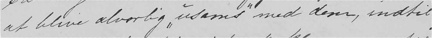at blive alvorlig "usams" med dem, indtil
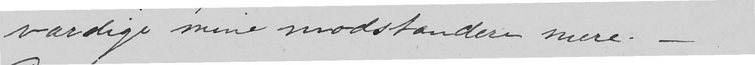værdige mine modstandere mere. -
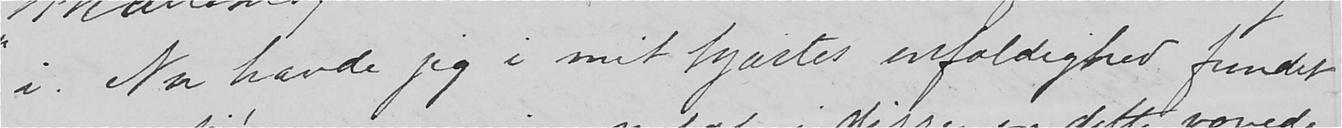i. Nu havde jeg i mit hjærtes enfoldighed fundet
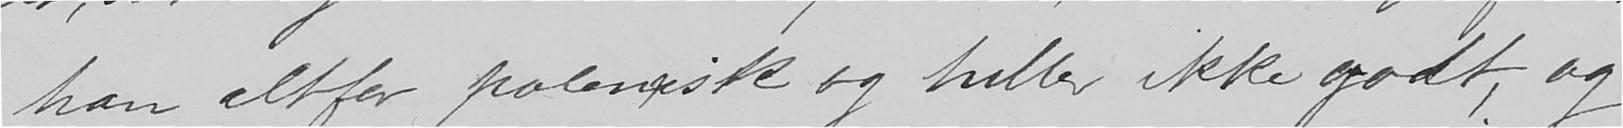han altfor polemisk og heller ikke godt, og
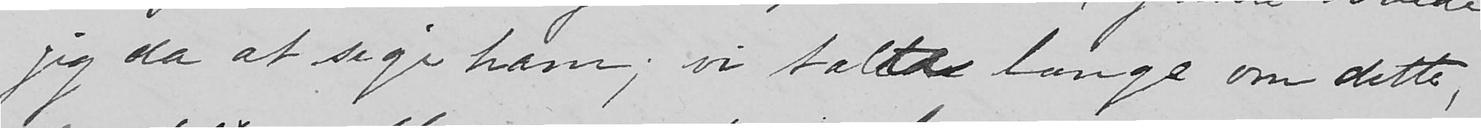jeg da at sige ham; vi talte længe om dette,
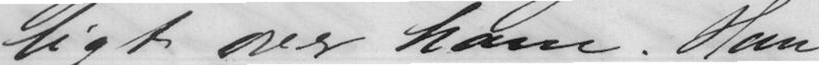ligt over ham. Han
